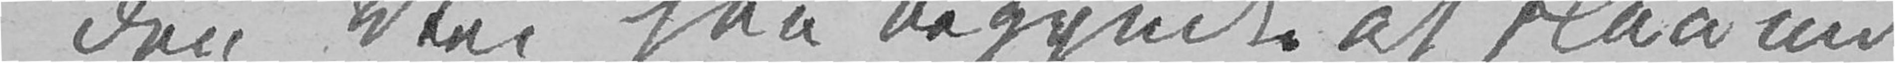den Vei han begyndte at slaa ind
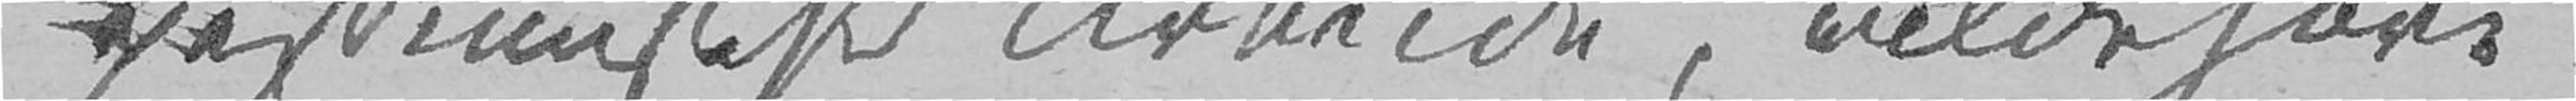pessimistisk Arbeide, vilde have
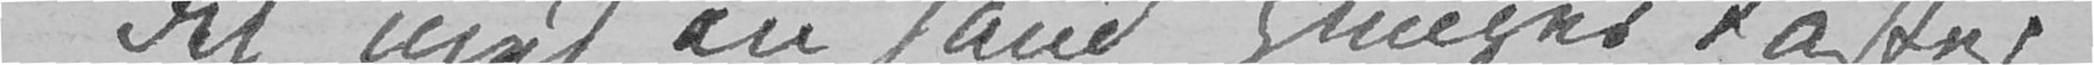det med en sand Hunger kaster
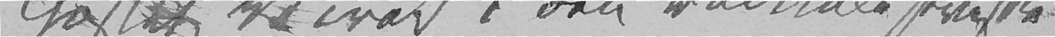høstet Virak i den radikale Presse
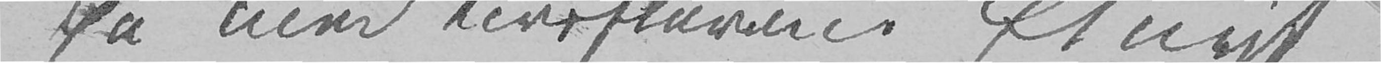på med «Livsslaven»[.] Et nyt
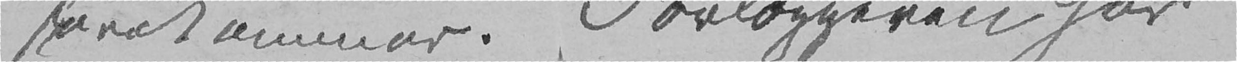forekommer. Forlæggeren har
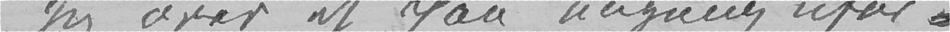sig over et paa engang ufor¬
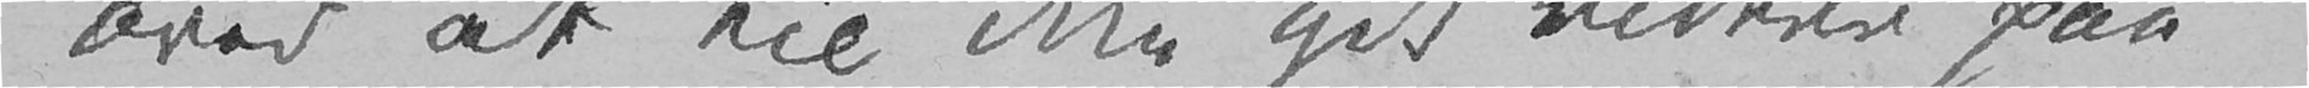over at Lie ikke gik videre paa
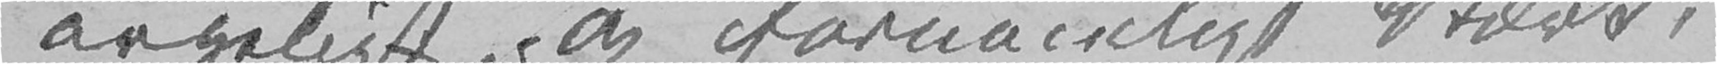argeligt og fornøieligt Værk,
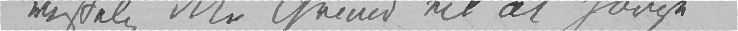visselig ikke Grund til at sørge
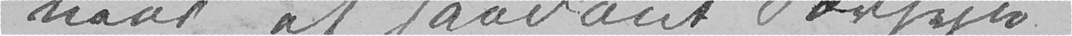naar et saadant Særsyn
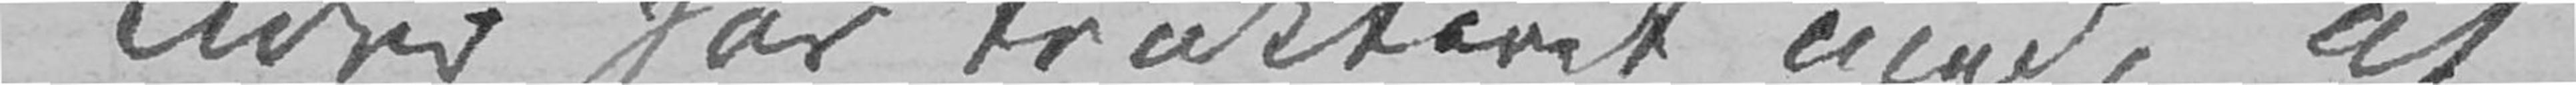Tider har trakteret med, at
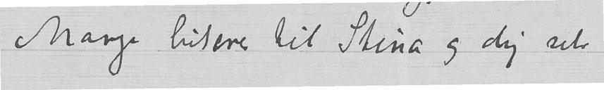Mange hilsener til Stina og dig selv
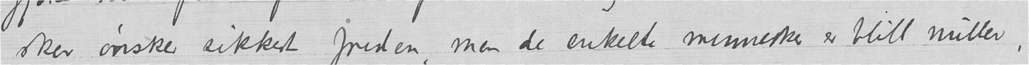sker ønsker sikkert freden, men de enkelte mennesker er blitt nuller
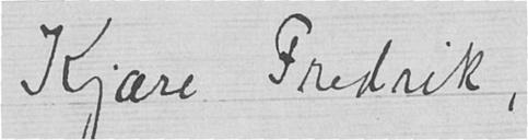Kjære Fredrik,
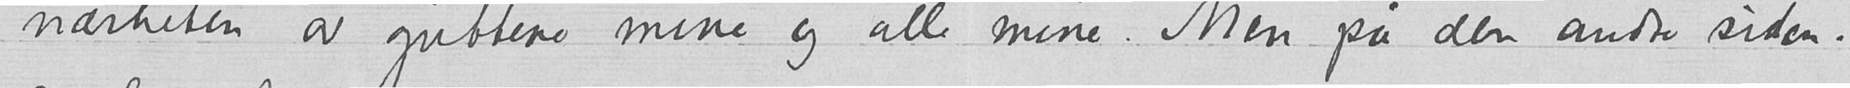nærheten av guttene mine og alle mine. Men på den andre siden.
